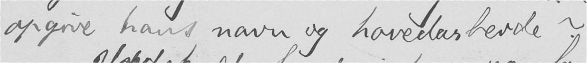opgive hans navn og hovedarbeide?
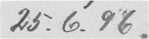25. 6. 96.
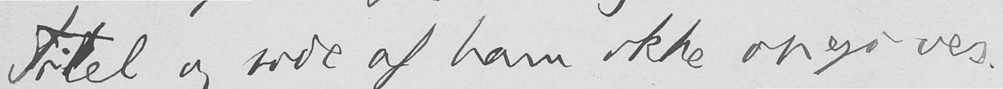Titel og side af ham ikke opgives.
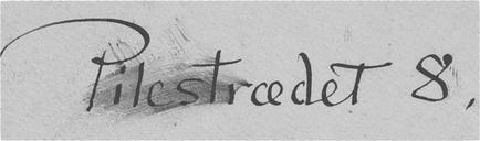Pilestrædet 8.
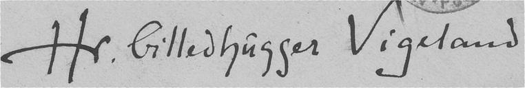Hr. billedhugger Vigeland
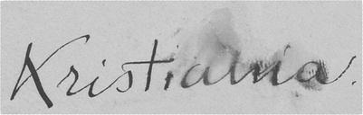Kristiania.
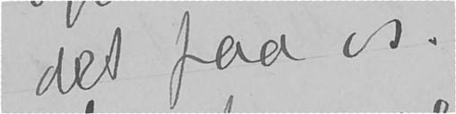det paa os.
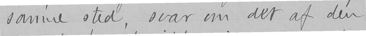samme sted, svar om det af den
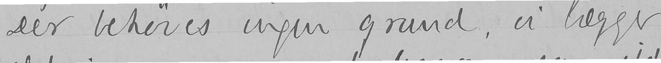Der behøves ingen grund, vi lægger
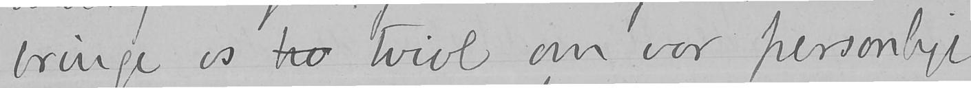bringe os ho tvivl om vor personlige
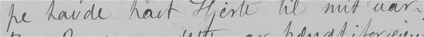pe havde havt Hjerte til mit uar-
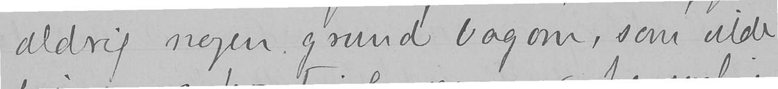aldrig nogen grund bagom, som vilde
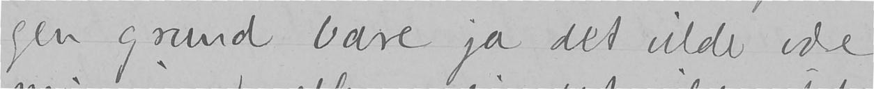gen grund bare ja det vilde være
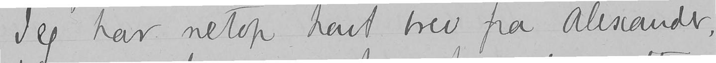Jeg har netop havt brev fra Alexander,
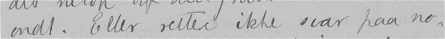ondt. Eller rettere ikke svar paa no-
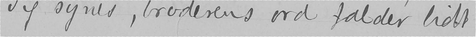Jeg synes, broderens ord falder lidt
